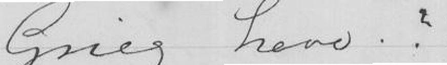Grieg have?
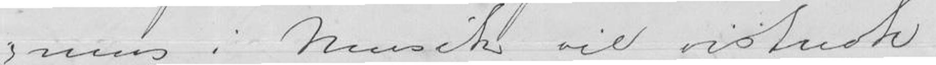ning i Musik vil vistnok
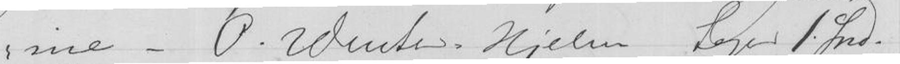me - O. Winter-Hjelm tager 1 spd.
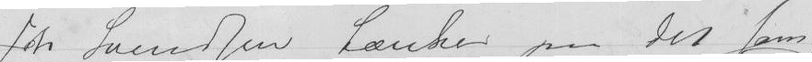Joh Svendsen tænker paa det sam-
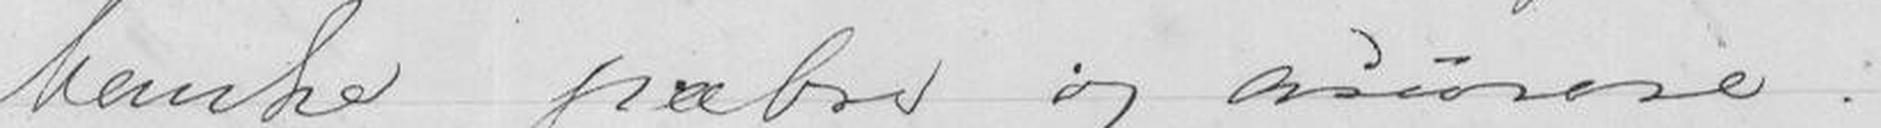bænke pæbre og assurere.
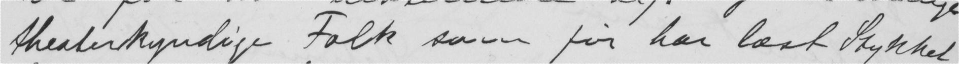theaterkyndige Folk som for har læst Stykket
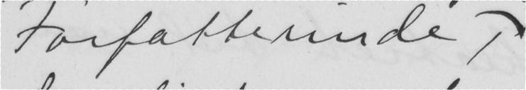Forfatterinde,
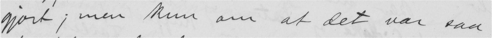gjort; men kun om at det var saa
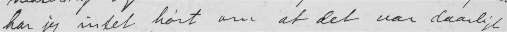har jeg intet hørt om at det var daarligt
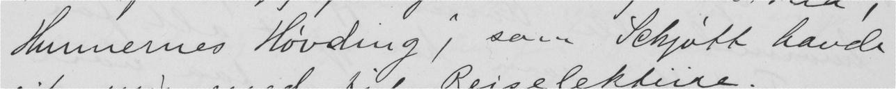Hunnernes Høvding", som Schjøtt havde
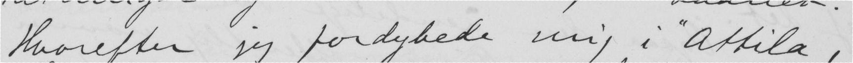Hvorefter jeg fordybede mig i "Attila,
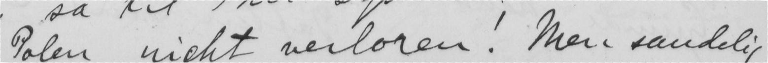Polen nicht verloren! Men sandelig
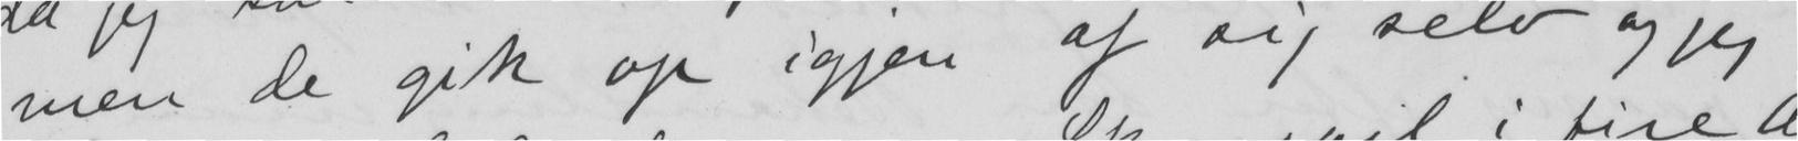men de gik op igjen af sig selv og jeg
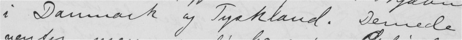i Danmark og Tyskland. Dernede
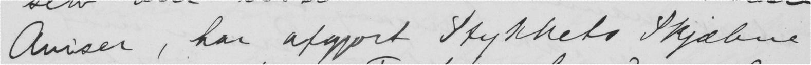Aviser, har afgjort Stykkets Skjæbne
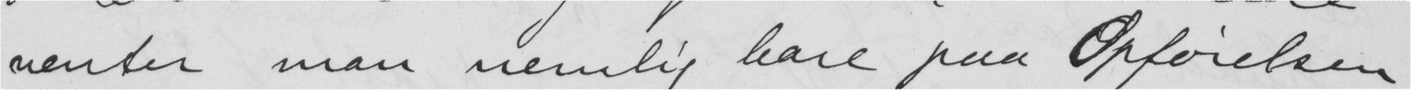venter man nemlig bare paa Opførelsen
